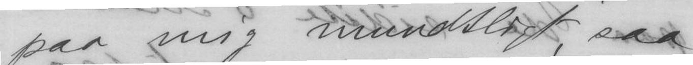paa mig mundtligt, saa
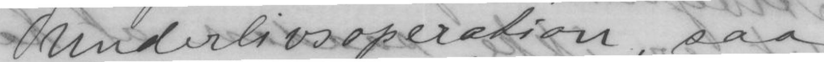Underlivsoperation, saa
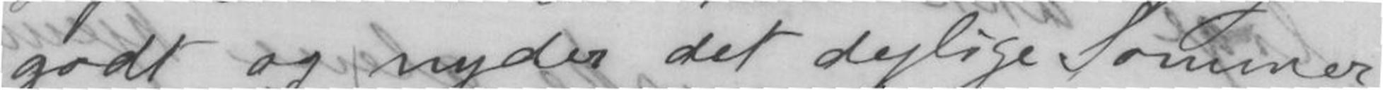godt og nyder det dejlige Sommer-
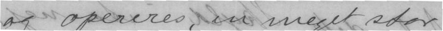4/ og opereres, en meget stor
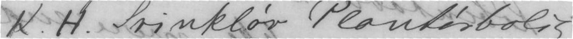K.H. Svinkløv Plantørbolig.
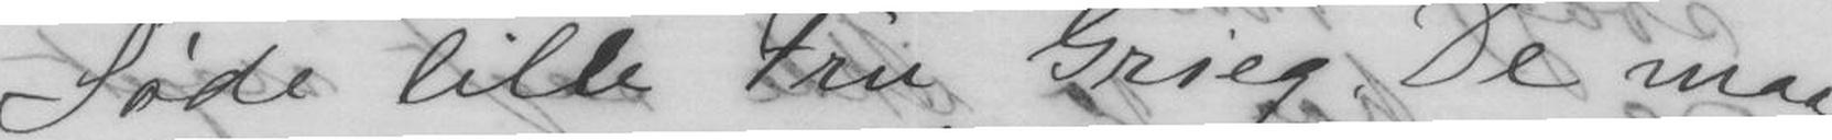Søde lille Fru Grieg De maa
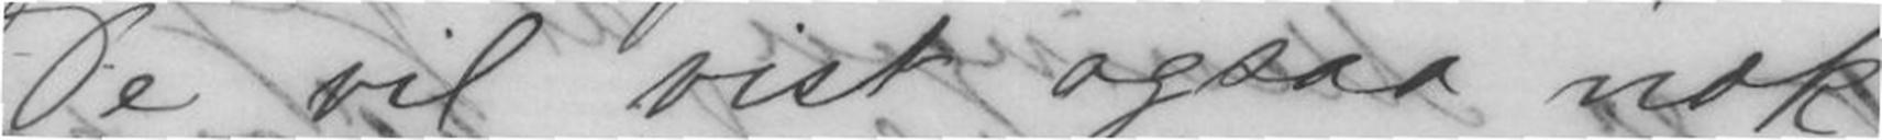De vil vist ogsaa nok
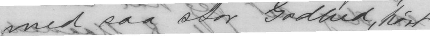med saa stor Godhed, hørt
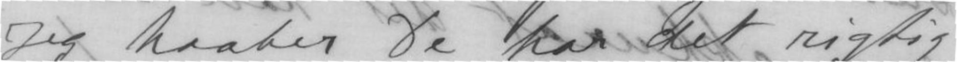Jeg haaber De har det rigtig
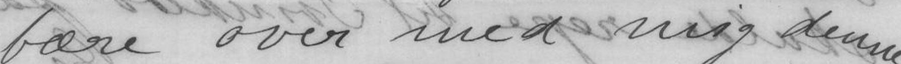bære over med mig denne
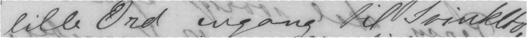lille Ord engang til Svinkløv
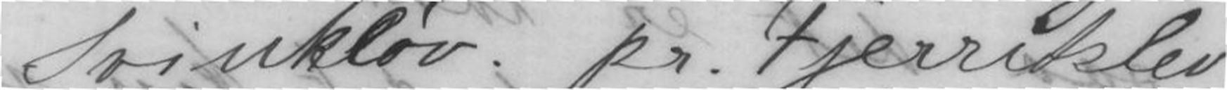Svinkløv pr. Fjerritslev.
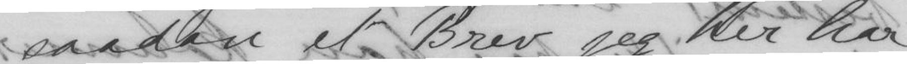saadane et Brev jeg her har
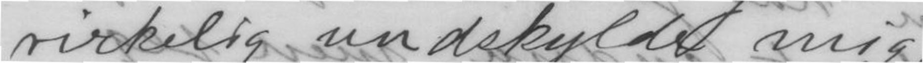virkelig undskylde mig
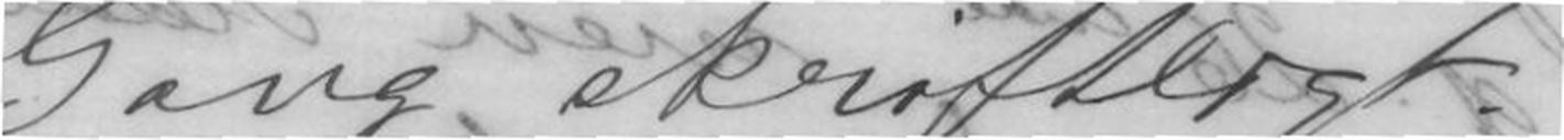Gang skriftligt.
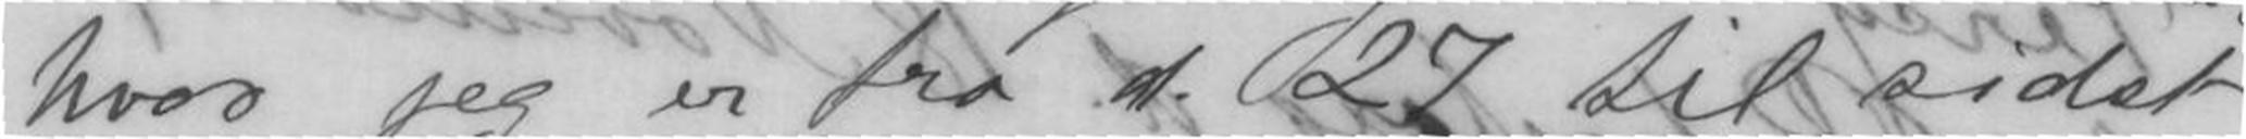hvor jeg er fra 27 til sidst
